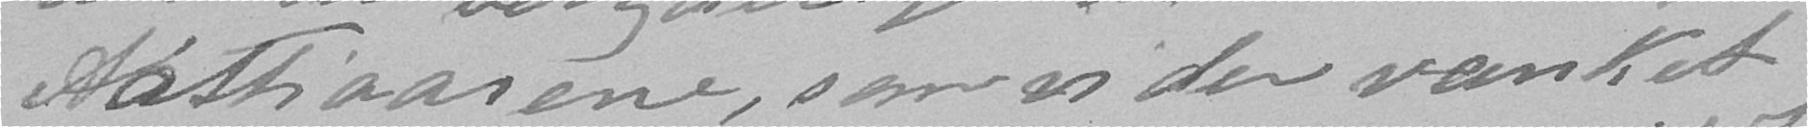Aattiaarene, som vi der vanket
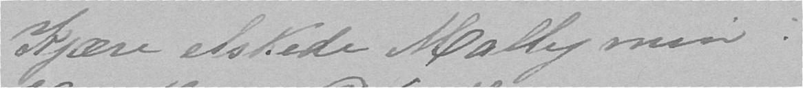Kjære elskede Mally min.
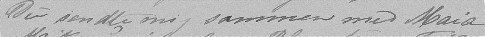Du sendte mig sammen med Maia
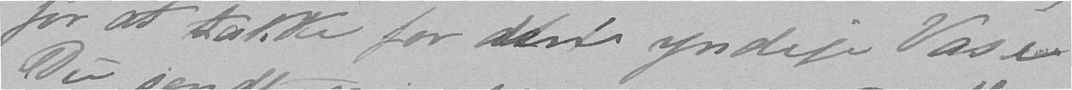for at takke for den yndige Vase
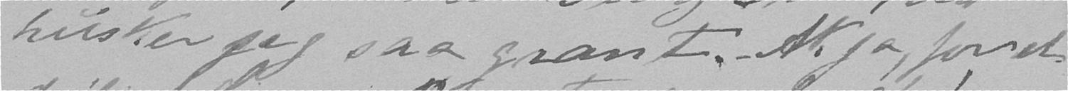husker jeg saa grant. Ak ja, for et
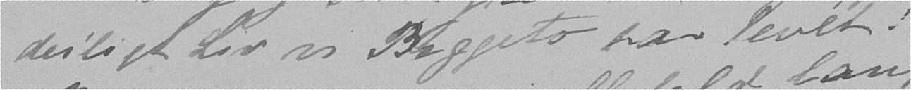deiligt Liv vi Beggeto har levet!
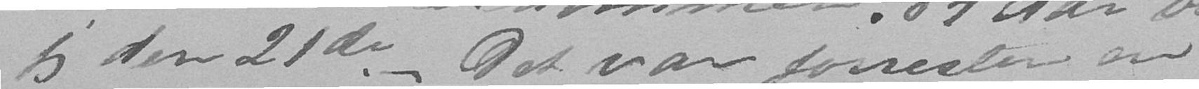jeg den 21de. - Det var forresten en
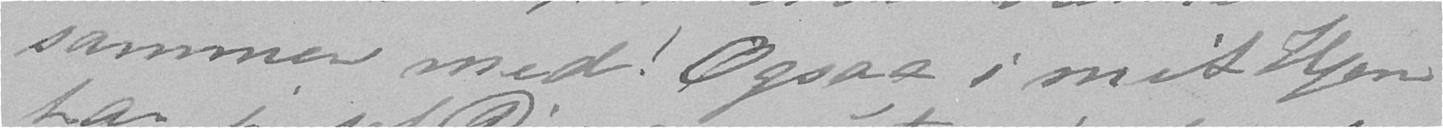sammen med. Ogsaa i mit Hjem
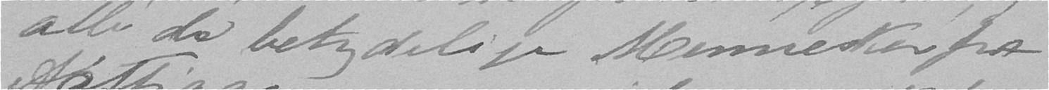alle de betydelige Mennesker fra
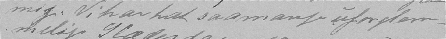mig. Vi har hat saamange uforglem-
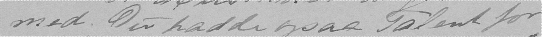med. Du hadde ogsaa Talent for
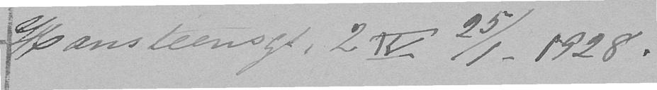Hansteensgt. 2 IV 25/1. 1928.
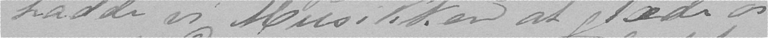hadde vi Musikken at glæde os
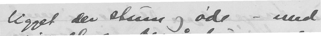ligget der stum og øde - med
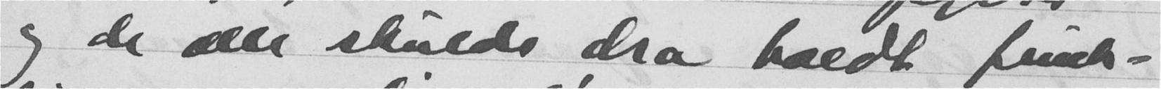og de alle skulde dra holdt funk-
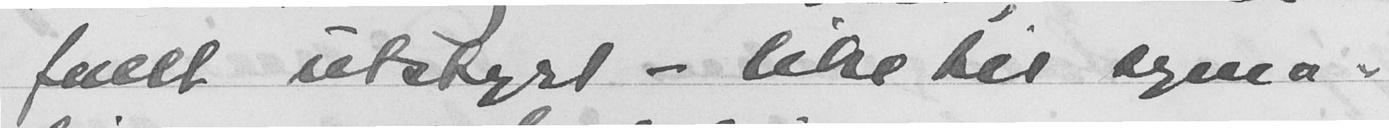fullt utstyrt - like til regne-
